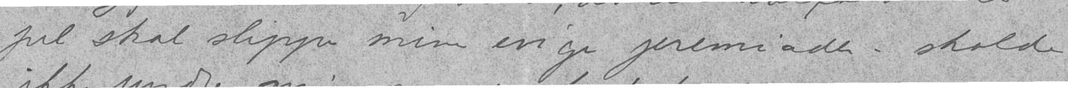jul skal slippe mine evige jeremiader — skulde
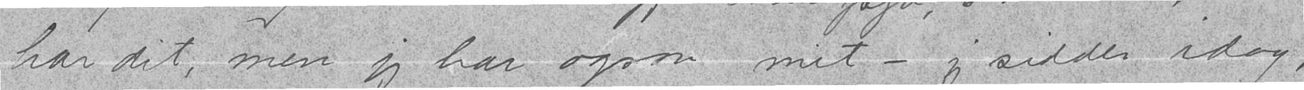har dit, men jeg har ogsaa mit — jeg sidder idag,
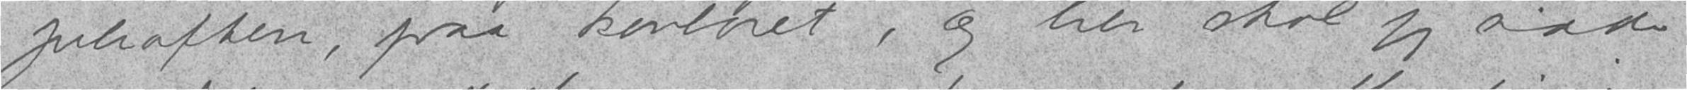julaften, paa kontoret, og her skal jeg sidde
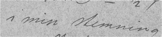i min stemning!
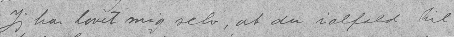Jeg har lovet mig selv, at du ialfald til
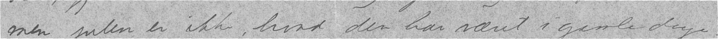men julen er ikke, hvad den har været i gamle dage.
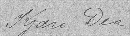Kjære Dea!
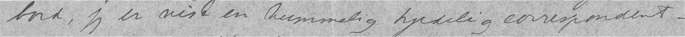bord, jeg er vist en temmelig kjedelig correspondent —
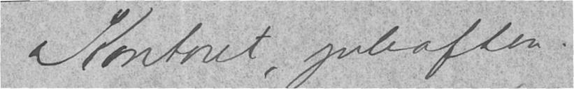Kontoret, juleaften.
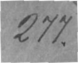277.
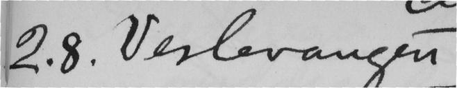2.8. Veslevangen
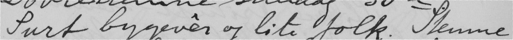Surt bygevêr og lite folk. Stemne
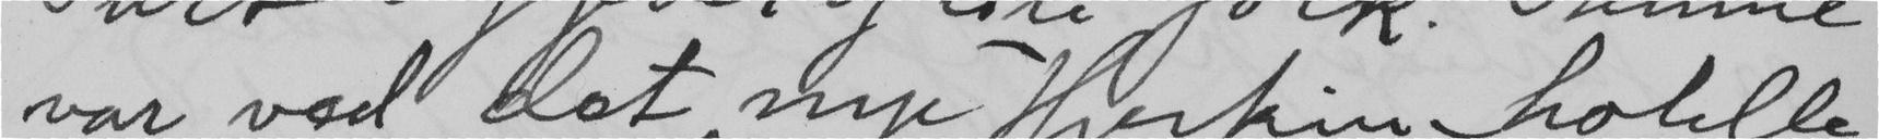var ved det nye Hjerkin-hotelle
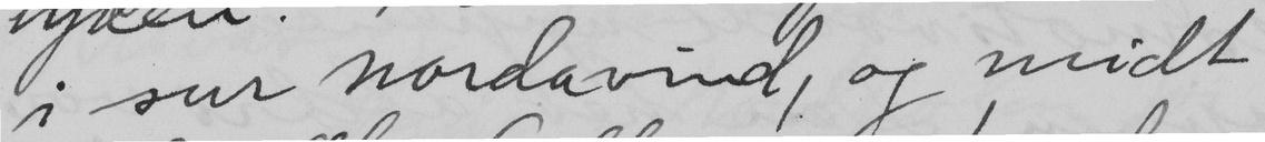i sur nordavind, og midt
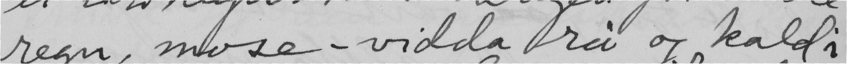regn, mose-vidda frå og kald i
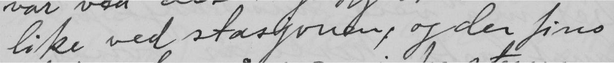like ved stasjonen, og der fins
